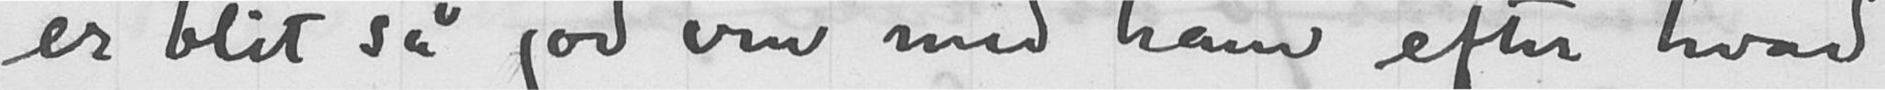er blit så god ven med ham efter hvad
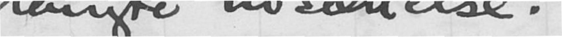langte udsættelse.
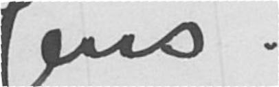Jens
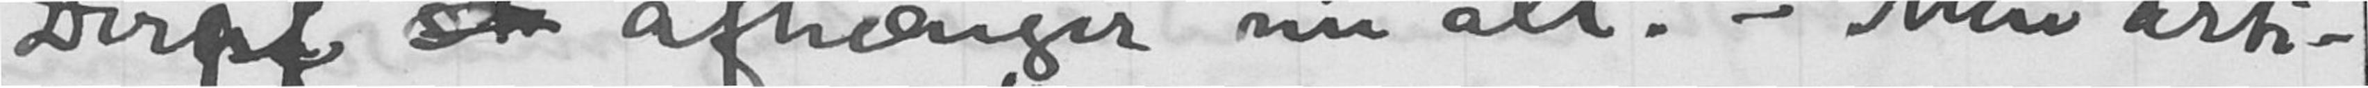Deraf st afhænger nu alt. - Min arti-
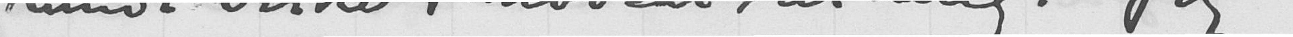kunde virke i modsat retning. Jeg
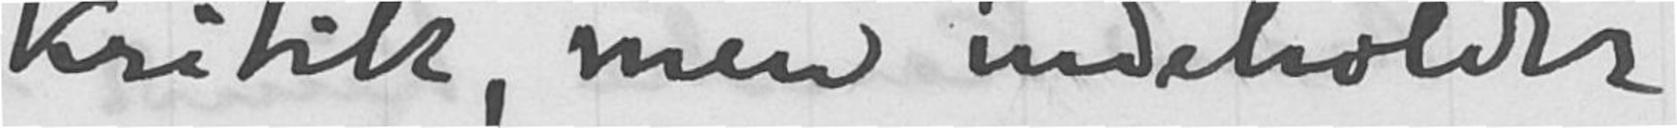kritik, men indeholder
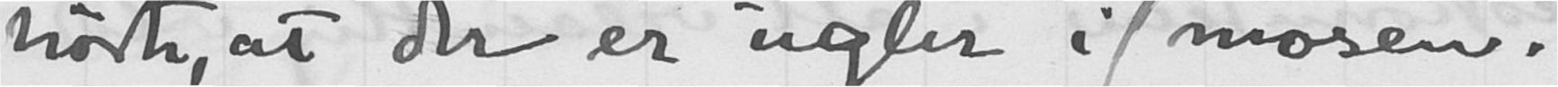hørte, at der er ugler i mosen.
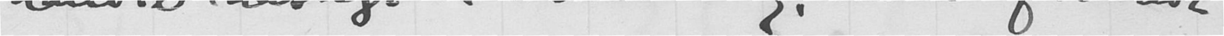handle hastigt i denne sag, men forberede
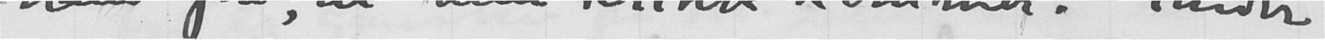ham på, at min kritik kommer. Finder
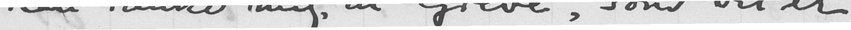kan tænke mig, at Greve, som vel er
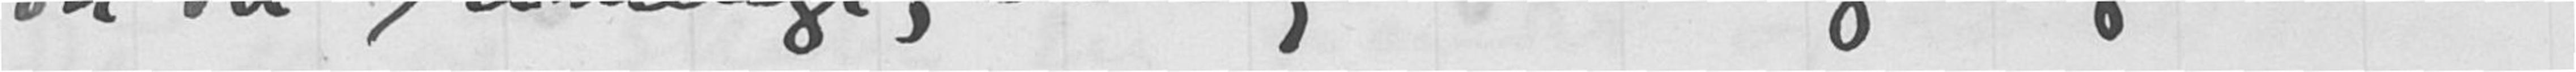du det rimeligt, så sig ham også grunden
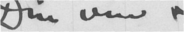Din ven
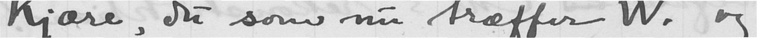Kjære, du som nu træffer W. og
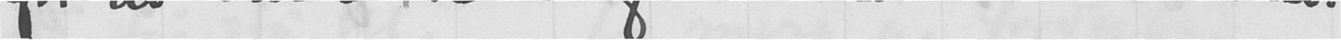for at vinde ham for vort vor modstand.
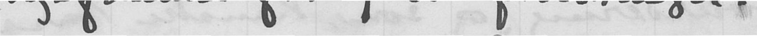ligefremme forslag til forbedringer.
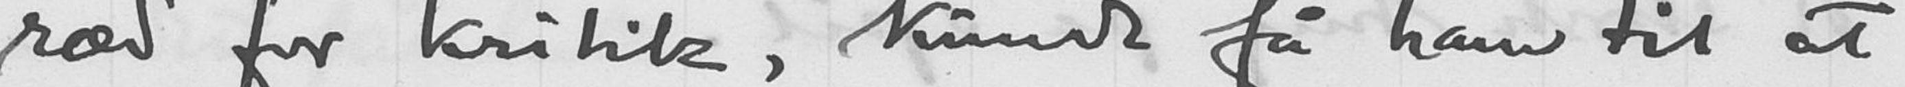ræd for kritik, kunde få ham til at
